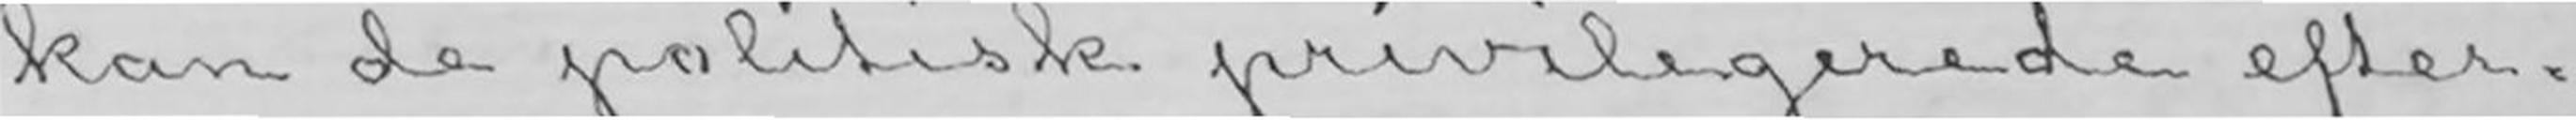kan de politisk priviligerede efter-
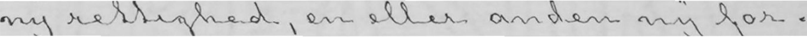ny rettighed, en eller anden ny for-
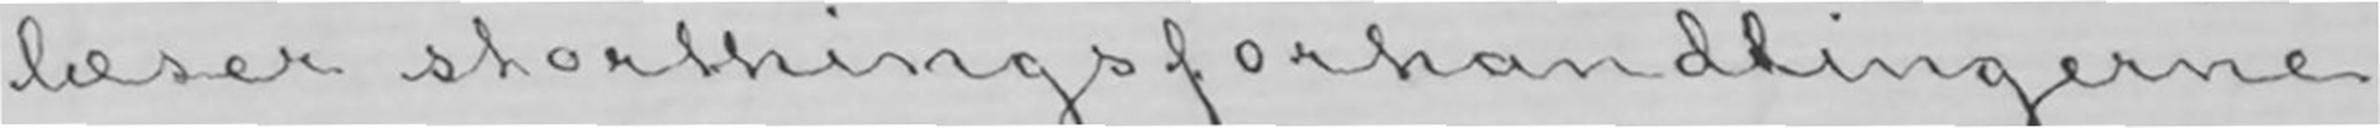læser storthingsforhandlingerne
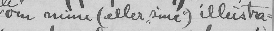om mine (eller "sine") illustra-
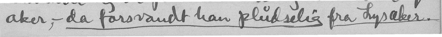aker, - da forsvandt han pludselig fra Lysaker.
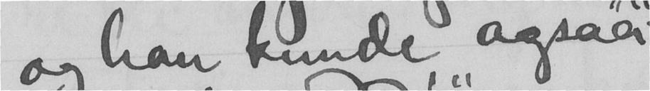og han kunde ogsaa
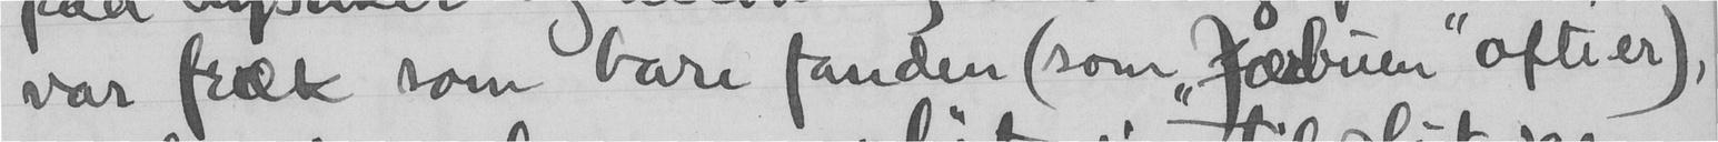var fræk som bare fanden (som "Jærbuen" ofte er),
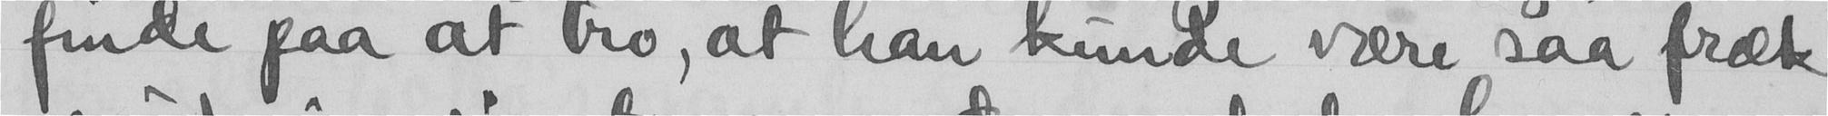finde paa at tro, at han kunde være saa fræk
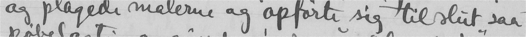og plagede malerne og opførte sig tilslut, saa
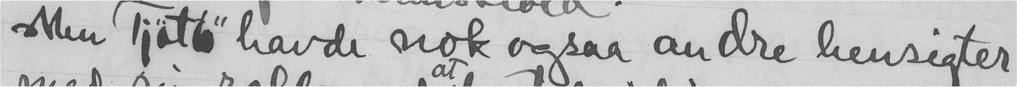Men Tjøttø havde nok ogsaa andre hensigter
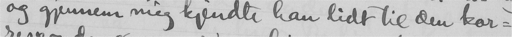og gjennem mig kjendte han lidt til den kor-
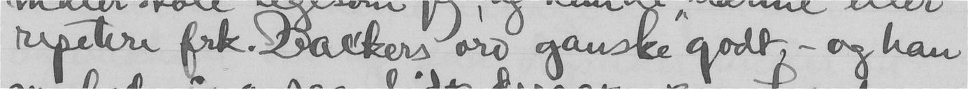repetere frk. Backers ord ganske godt, - og han
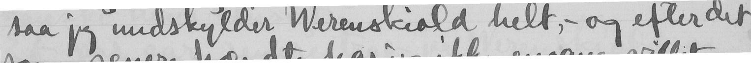saa jeg undskylder Werenskiold helt, - og efter det
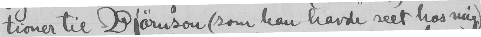tioner til Bjørnson (som han havde seet hos mig)
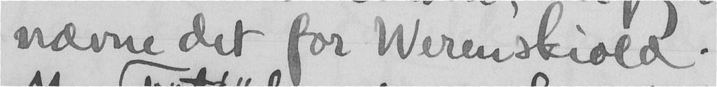nævne det for Werenskiold.
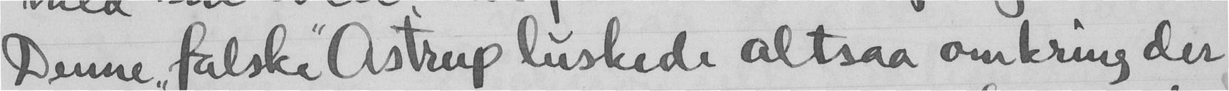Denne "falske" Astrup luskede altsaa omkring der
